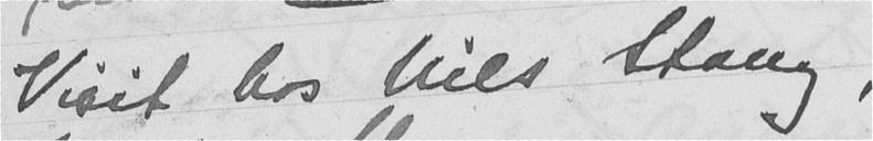Visit hos Nils Stang,
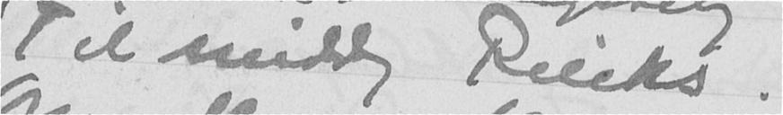Til middag Riecks.
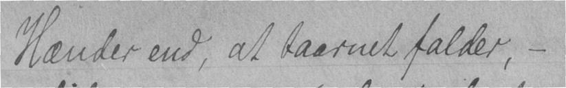Hænder end, at taarnet falder, -
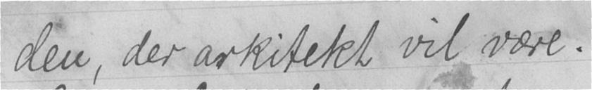den, der arkitekt vil være.
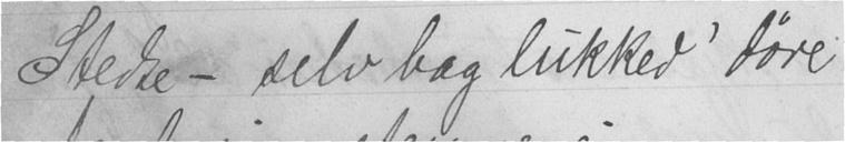Stedse - selv bag lukked' døre
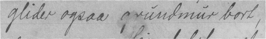glider ogsaa grundmur bort,
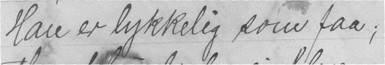Han er lykkelig som faa;
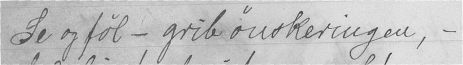Se og føl - grib ønskeringen, -
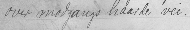over modgangs haarde vei.
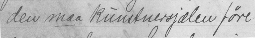den maa kunstnersjælen føre
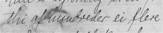thi af hundreder ei flere
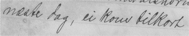næste dag, ei kom tilkort
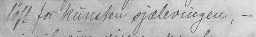løft for kunsten sjælevingen, -
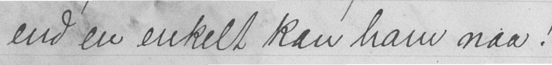end en enkelt kan ham naa!
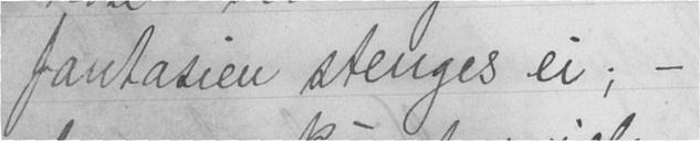fantasien stenges ei; -
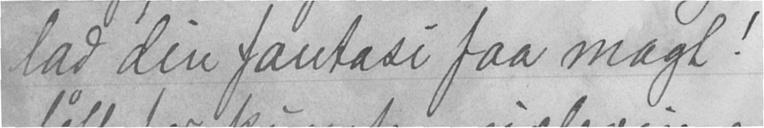lad din fantasi faa magt!
